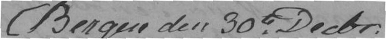Bergen den 30te Decbr.
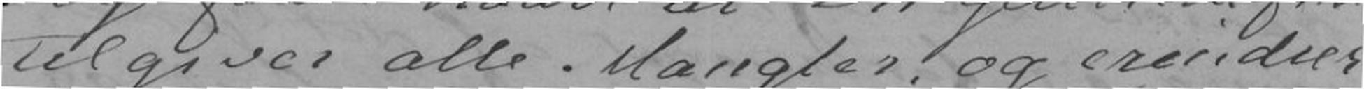tilgiver alle. Mangler og erindrer
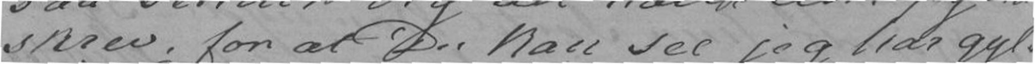skrev, for at Du kan see jeg har gyl-
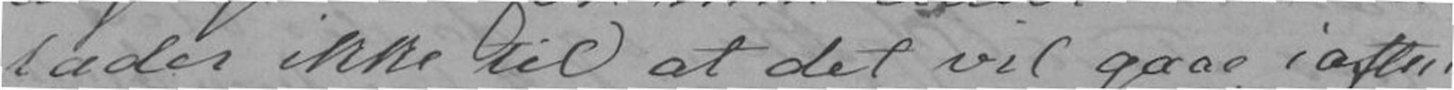lader ikke til at det vil gaae iaften
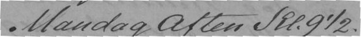Mandag Aften Kl 9½.
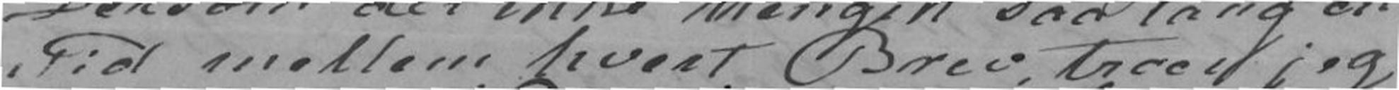Tid mellem hvert Brev, troer jeg
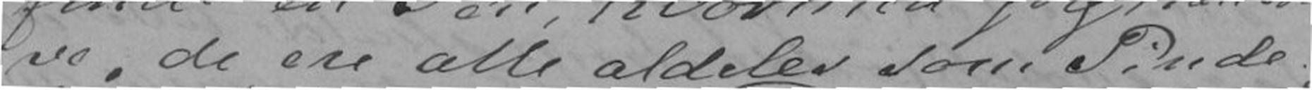ve, de ere alle aldeles som Pinde.
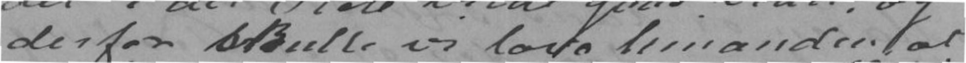derfor skulle vi love hinanden at
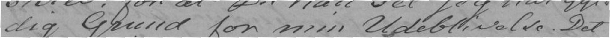dig Grund for min Udeblivelse. Det
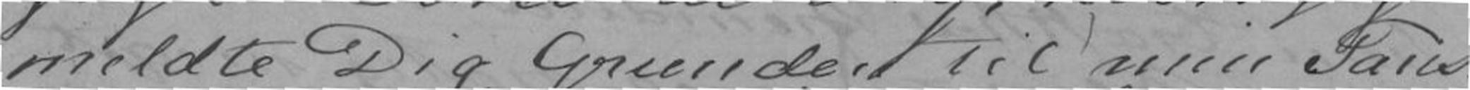meldte Dig Grunde til min Taus-
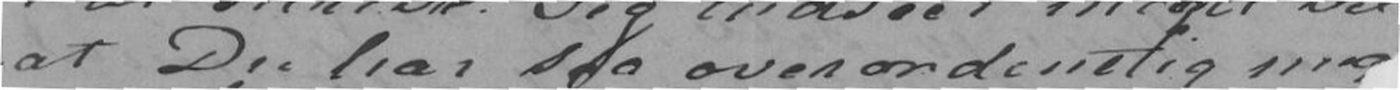at Du har saa overordentlig me
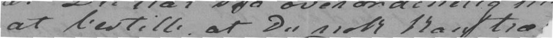at bestille at Du nok kan træ
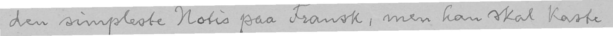den simpleste Notis paa Fransk, men han skal kaste
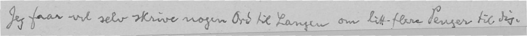Jeg faar vel selv skrive nogen Ord til Langen om litt flere Penger til dig.
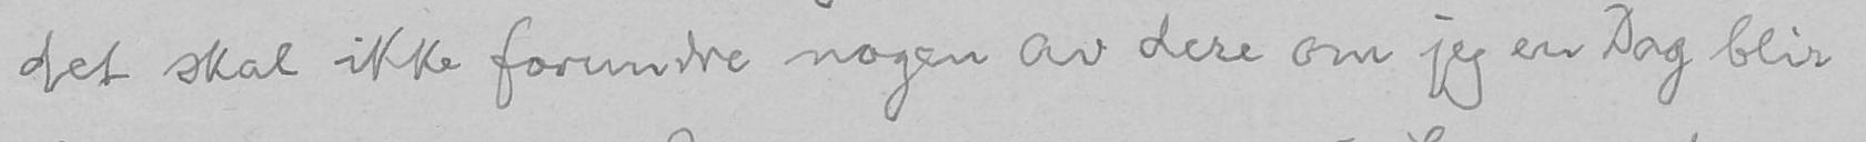det skal ikke forundre nogen av dere om jeg en Dag blir
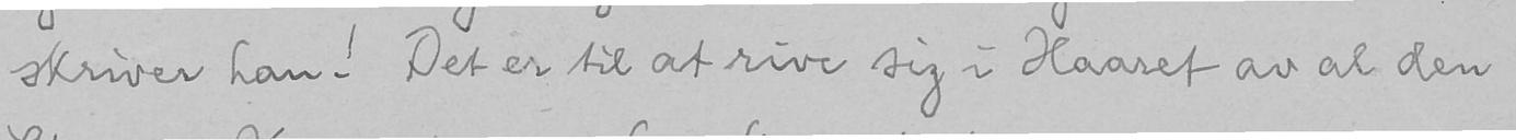skriver han! Det er til at rive sig i Haaret av al den
